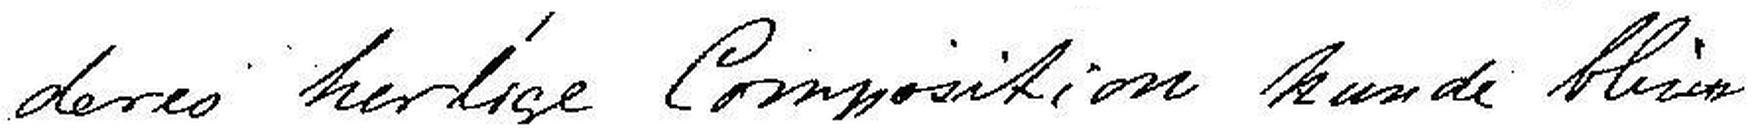deres herlige Composition kunde blive
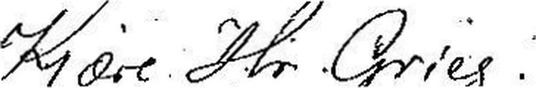Kjære Hr Grieg!
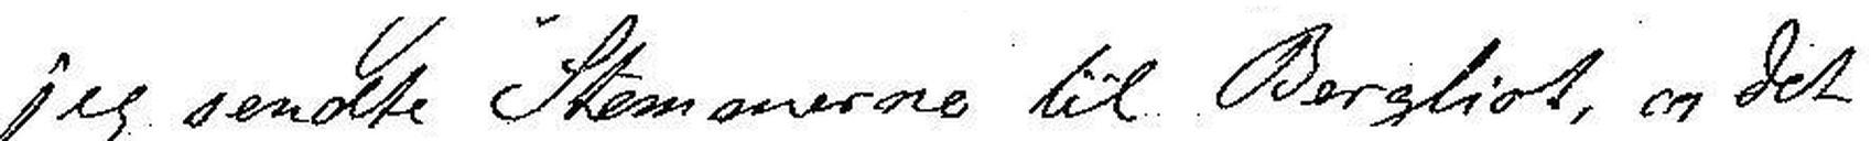jeg sendte Stemenerne til Bergliot, og det
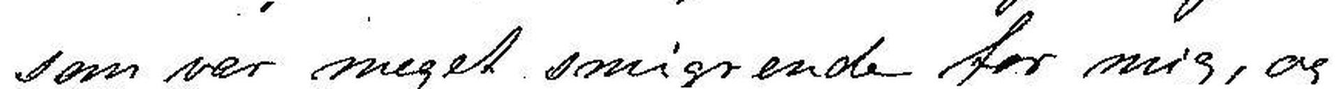som var meget smigrende for mig, og
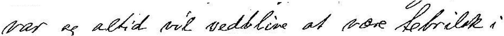var og altid vil vedblive at være febrilsk i
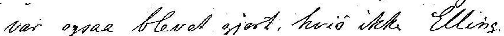var ogsaa bledet gjort, hvis ikke Elling
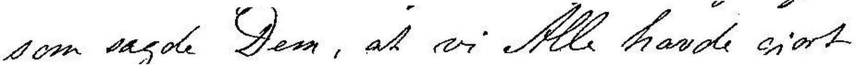som sagde Dem, at vi Alle havde gjort
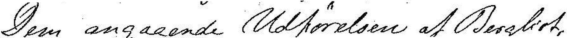Dem angaaende Udførelsen af Bergliot,
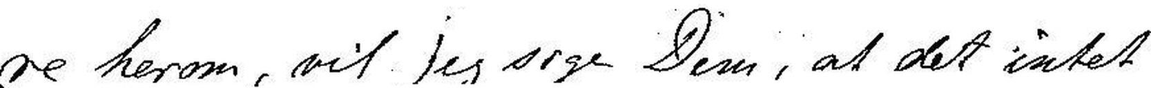re herom, vil jeg sige Dem, at det intet
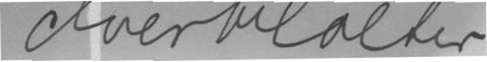Iver Holter
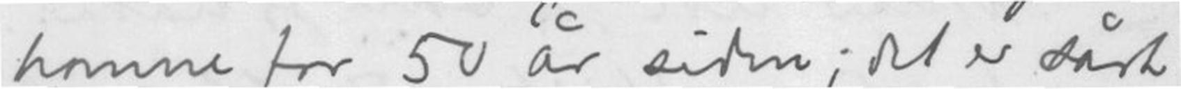komne for 50 år siden; det er sårt
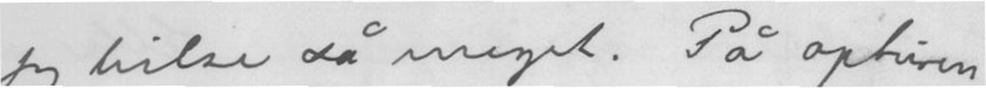jeg hilse så meget. På opturen
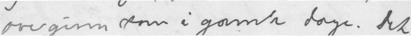overgiven som i gamle dage. Det
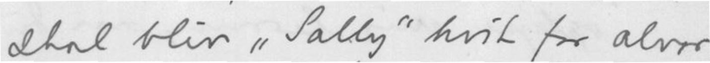skal blive "Sally " hvit for alvor.
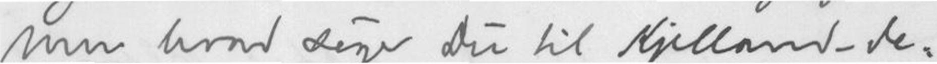Men hvad siger Du til Kjelland-de-
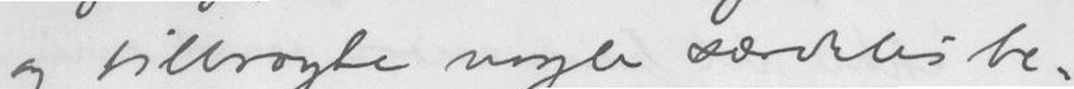og tilbragte nogle særdeles be-
